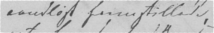aandløst fremstillede,
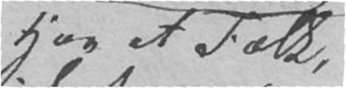Hos et Folk,
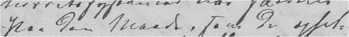Paa den Maade, som de opfat-
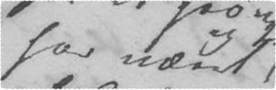har været
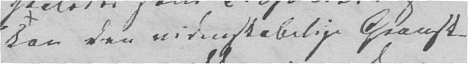kan den videnskabelige Gransk-
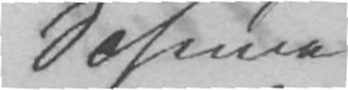Schema
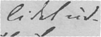lidet ud-
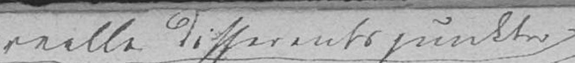reelle Differentspunkter
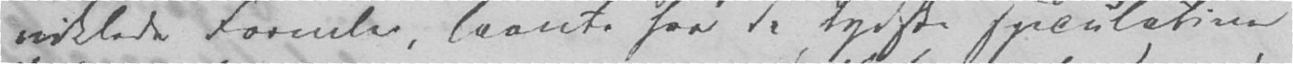viklede Formler, laante hos de tydske speculative
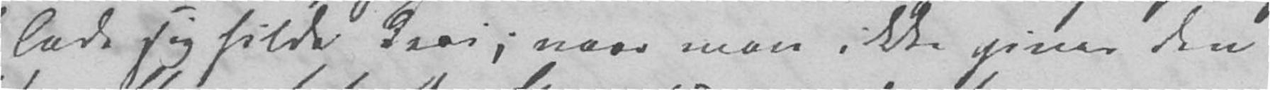lade sig hilde deri; naar man ikke giver den
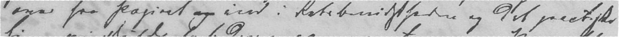over fra Papiret og ind i Retsbevidstheden og det practiske
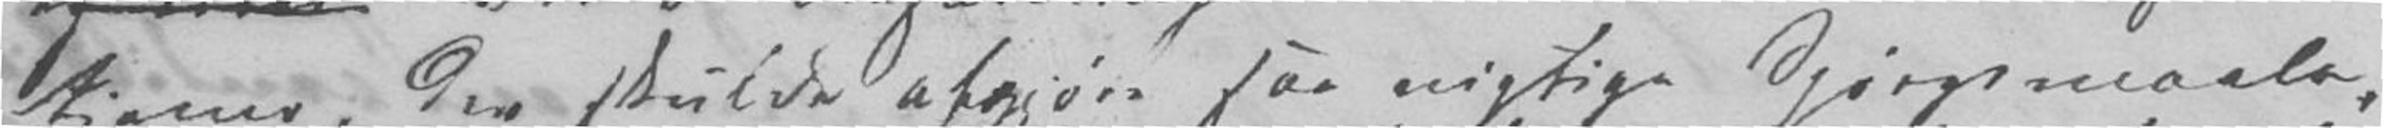tioner, der skulde afgjøre saa vigtige Spørgsmaale,
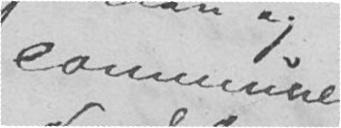commune
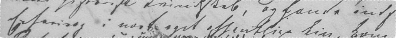Erfaring i vort eget offentlige Liv, kom
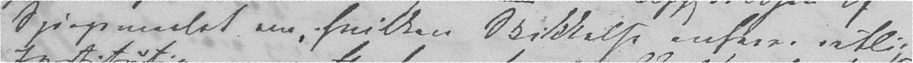Spørgsmaalet om, hvilken Skikkelse enhver retlig
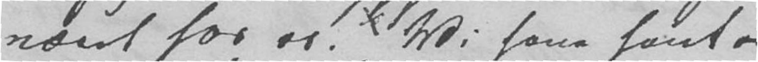været hos os. Vi have havt
